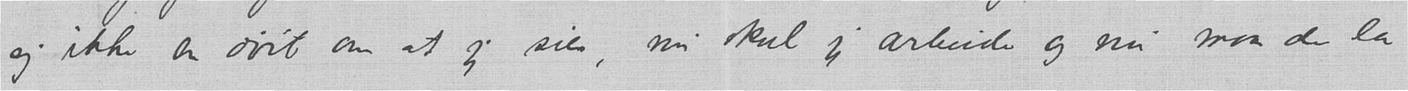sig ikke en døit om at jeg sier, nu skal jeg arbeide og nu maa de la
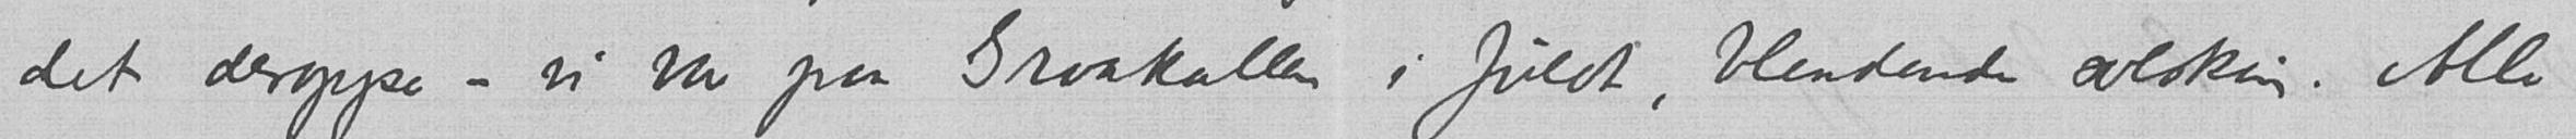det deroppe – vi var paa Graakallen i fuldt, blendende solskin. Alle
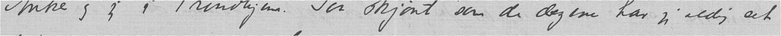Anker og jeg i Trondhjem. Saa skjønt som de dagene har jeg aldrig set
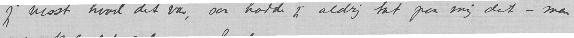jeg visst hvad det var, saa hadde jeg aldrig tat paa mig det - men
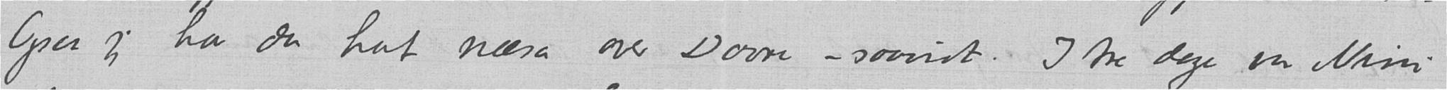Ogsaa jeg har da hat næsa over Dovre – saavidt. I tre dager var Nini
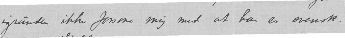igrunden ikke forsone mig med at han er svensk.
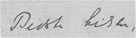Bedste hilsen,
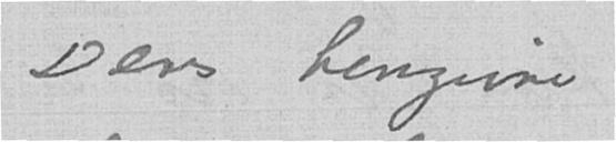Deres hengivne
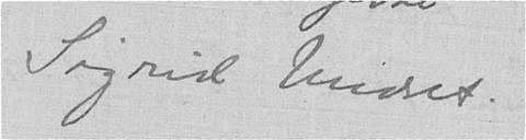Sigrid Undset.
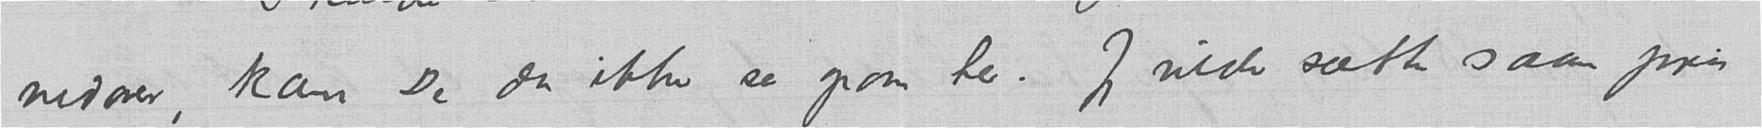nedover, kan De da ikke se opom her. Jeg vilde sætte saan pris
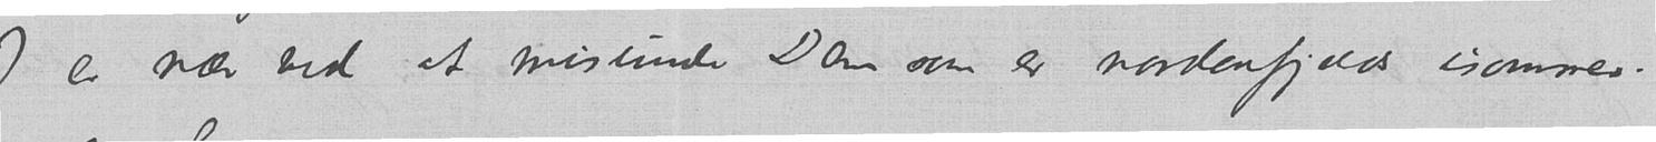Jeg er nær ved at misunde Dem som er nordenfjelds isommer.
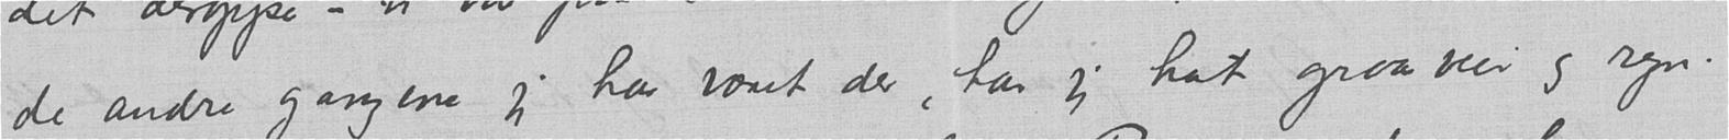de andre gangene jeg har været der, har jeg hat graaveir og regn.
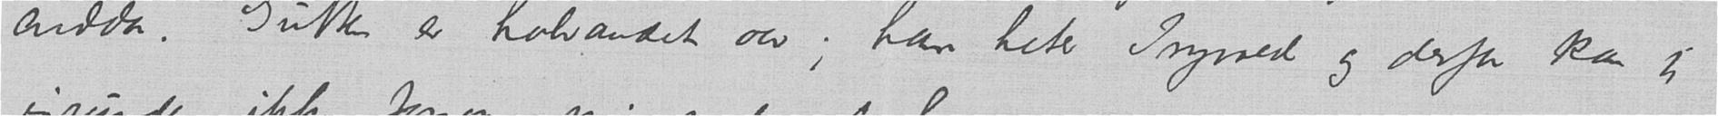endda. Gutten er halvandet aar; han heter Ingvald og derfor kan jeg
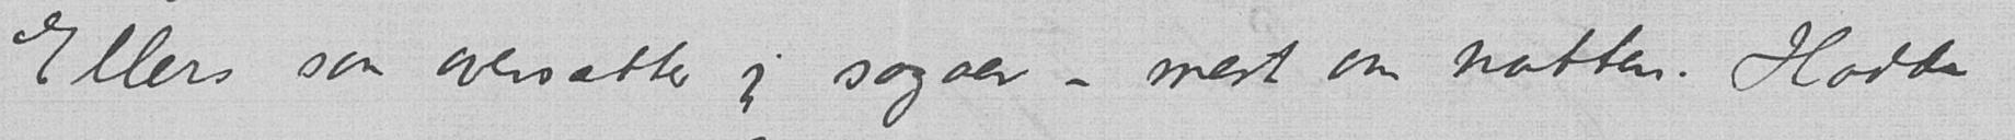Ellers saa oversætter jeg sagaer – mest om natten. Hadde
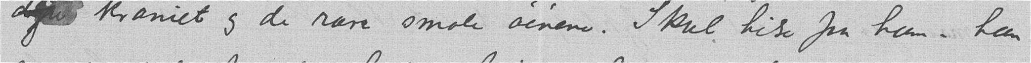kraniet og de rare smale øinene. Skal hilse fra ham – han
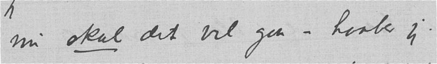nu skal det vel gaa – haaber jeg.
